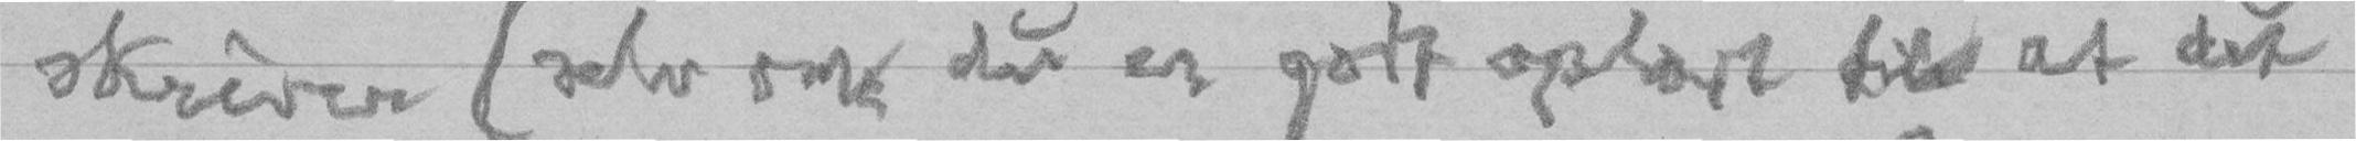skriver (selv om du er godt oplært til at det
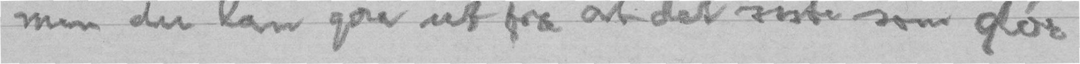men du kan gaa ut fra at det siste som dør
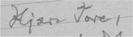Kjære Tore,
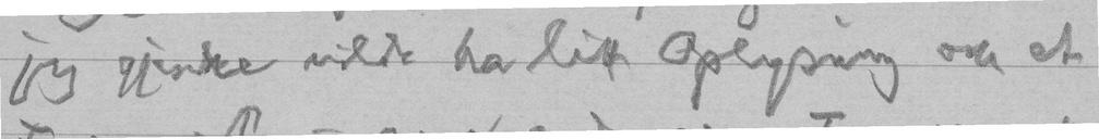jeg gjerne vilde ha litt Oplysning om et
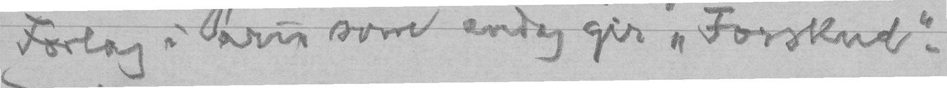Forlag i Paris som endog gir "Forskud".
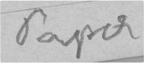Papa
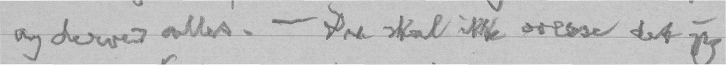og derved alles. - Du skal ikke overse det jeg
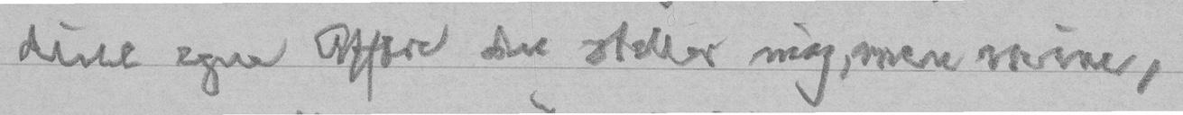dine egne Affærer du steller mig, men mine,
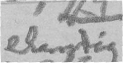elendig
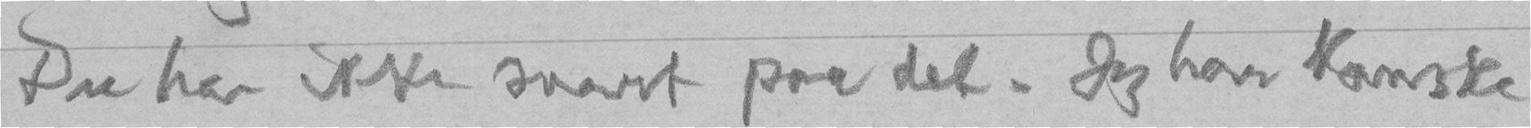Du har ikke svart paa det. Jeg har kanske
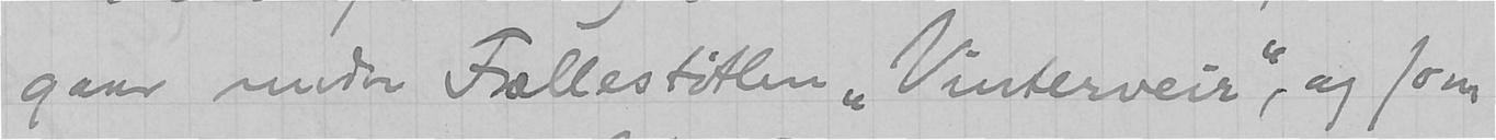gaar under Fællestitlen "Vinterveir", og som
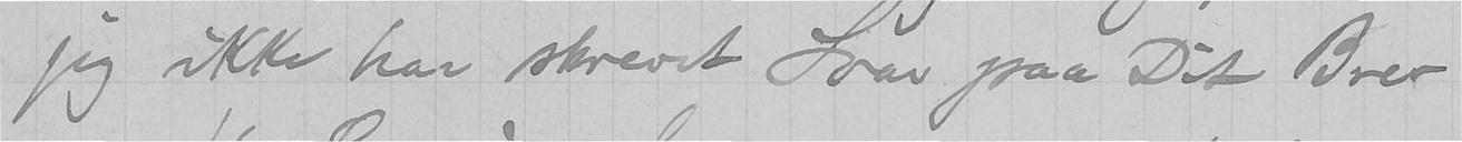jeg ikke har skrevet Svar paa Dit Brev
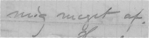mig meget af.
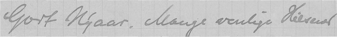Godt Nyaar. Mange venlige Hilsner
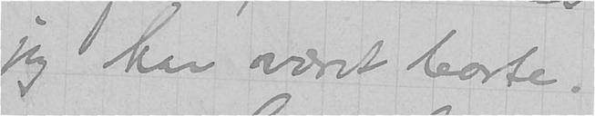jeg har været borte.
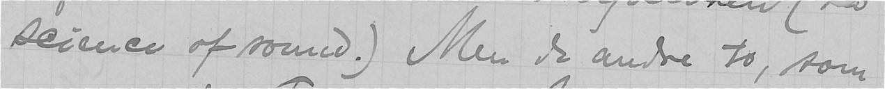science of sound.) Men de andre to, som
